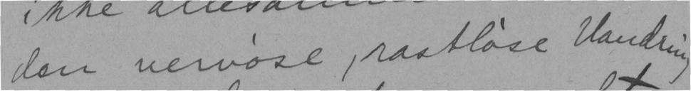den nervøse, rastløse Vandring
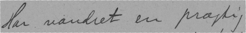Har vandret en prægtig
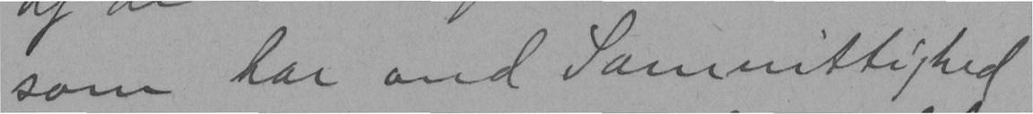som har ond Samvittighed
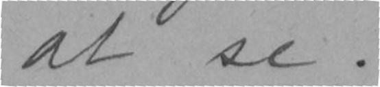at se.
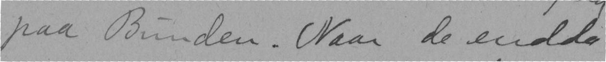paa Bunden. Naar de endda
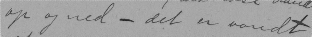op og ned - det er vondt
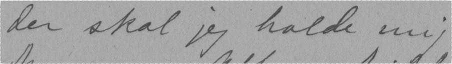der skal jeg holde mig
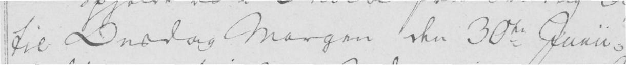til Onsdag Morgen den 30te Junii;
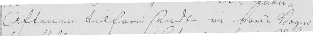Aftenen tilforn sendte vi vores Vogn
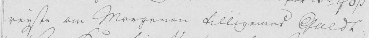reyste om Morgenen tilligemed Galdt
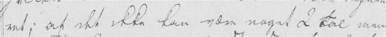ret; at det ikke kan være noget 2 Tal men
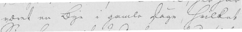været en Bye i gamle Dage, hvilket
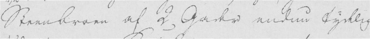Steenbroen af 2 Gader endnu tydelig
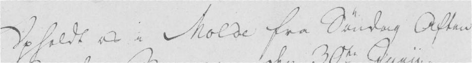Opholdt os i Molde fra Søndag Aften
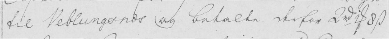til Veblungsnæs og betalte derfor 2 rd 48 s
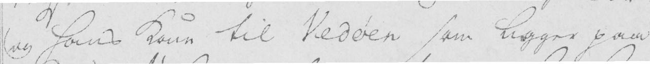og hans Kone til Vedøen som ligger paa
